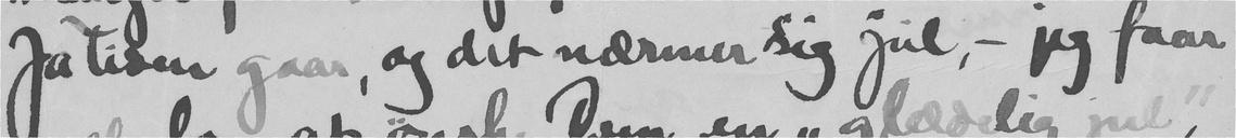Ja tiden gaar, og det nærmer sig jul, - jeg faar
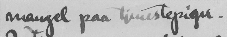mangel paa tjenestepiger.
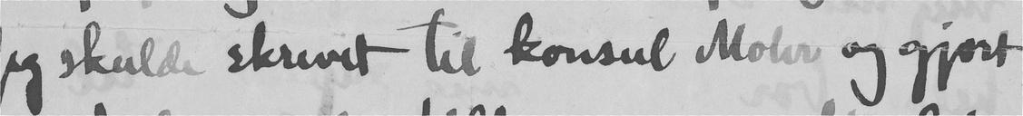Jeg skulde skrevet til konsul Mohr og gjort
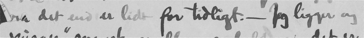a det end er lidt for tidligt. - Jeg ligger og
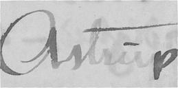Astrup
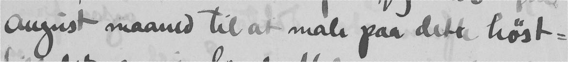august maaned til at male paa dette høst-
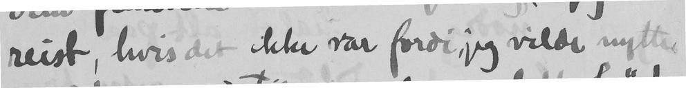reist, hvis det ikke var fordi, jeg vilde nytter
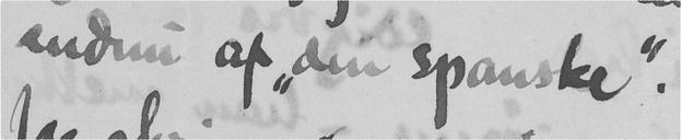endnu af "din spanske".
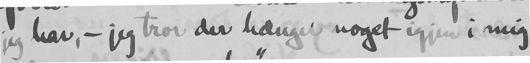jeg har, - jeg tror der hænger noget igjen i mig
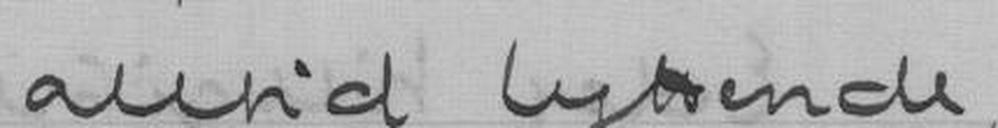alltid lyttende,
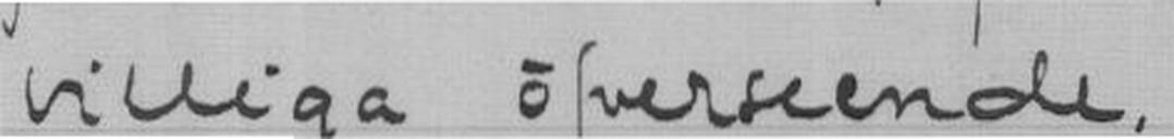villiga öfverseende.
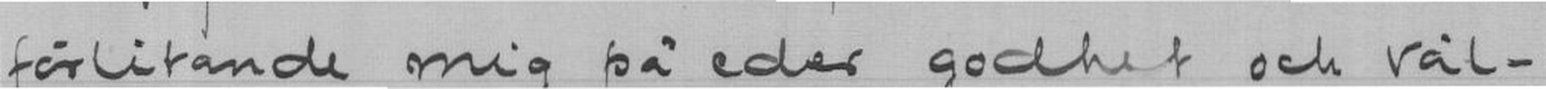förlitande mig på eder godhet och väl-
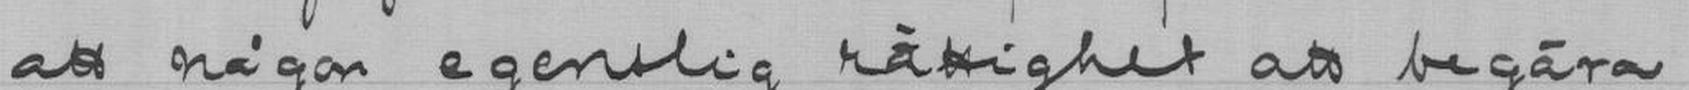att någen egentlig rättighet att begära
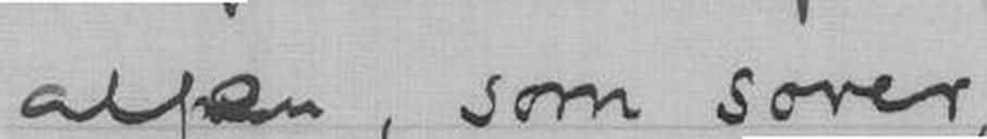alfen, som sover,-
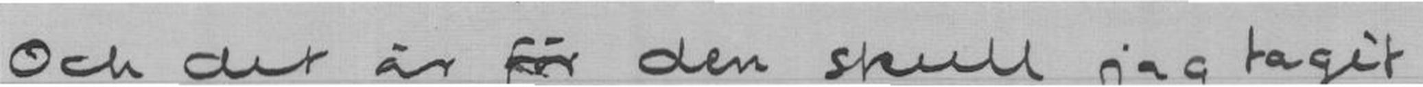Och der är för den skull jag tagit
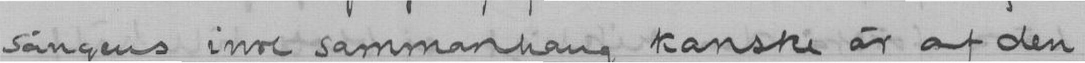sångens inre sammanhang kanske är af den
